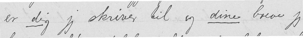er dig jeg skriver til og dine breve jeg
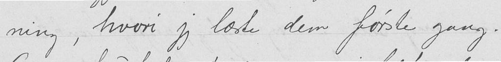ning, hvori jeg læste dem første gang.
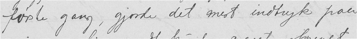første gang, gjorde det mest indtryk paa
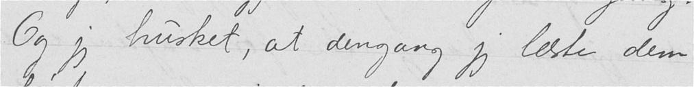Og jeg husket, at dengang jeg læste dem
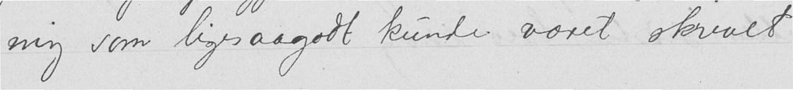mig som ligesaagodt kunde været skrevet
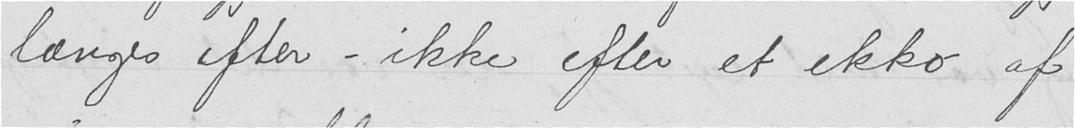længes efter - ikke efter et ekko af
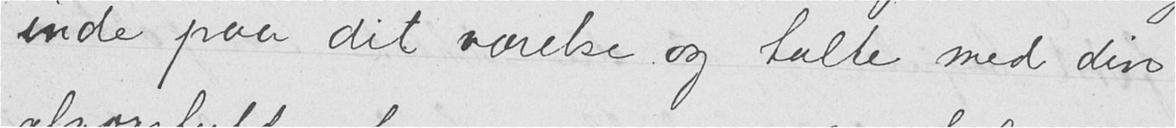inde paa dit værelse og talte med din
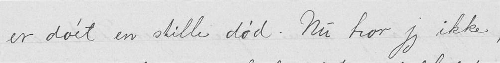er døet en stille død. Nu har jeg ikke,
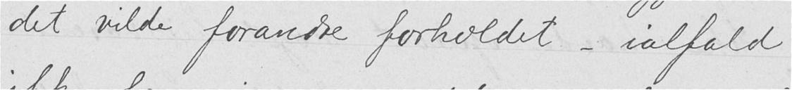det vilde forandre forholdet - ialfald
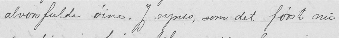alvorsfulde øine. Jeg synes, som det først nu
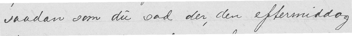saadan som du sad der, den eftermiddag
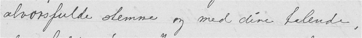alvorsfulde stemme og med dine talende,
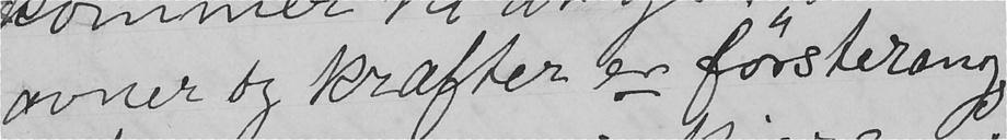ævner og kræfter er førsterangs.
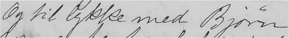Og til lykke med Bjørn
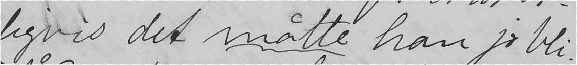ligvis det måtte han jo bli.
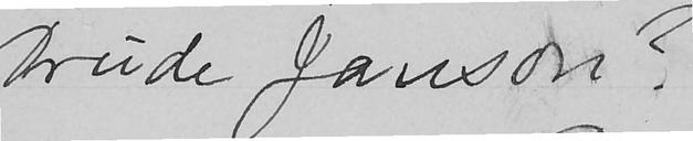Drude Janson?
